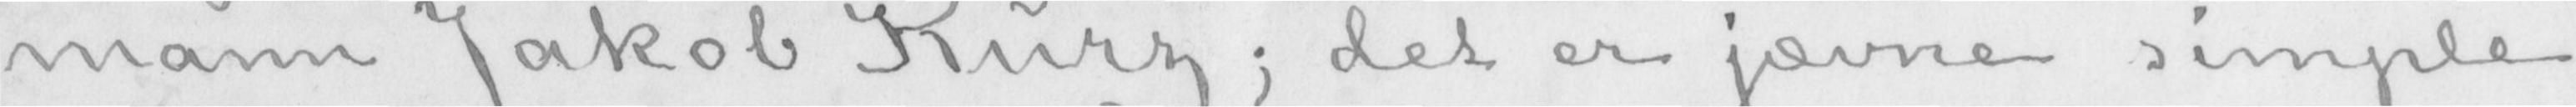mann Jakob Kurz; det er jævne simple
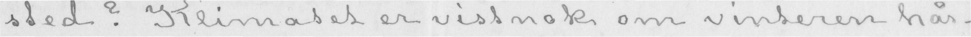sted? Klimatet er vistnok om vinteren hår-
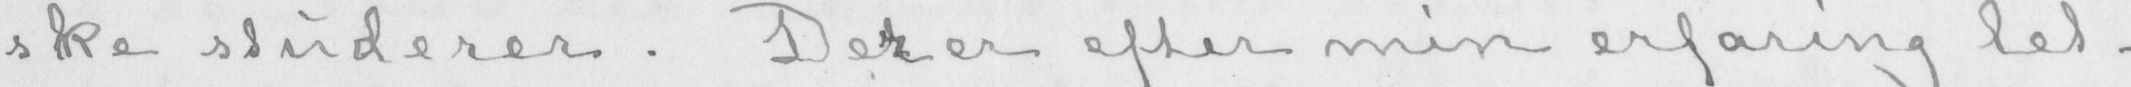ske studerer. Der er efter min erfaring let-
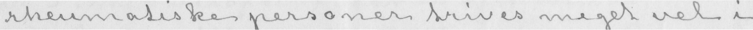rheumatiske personer trives meget vel i
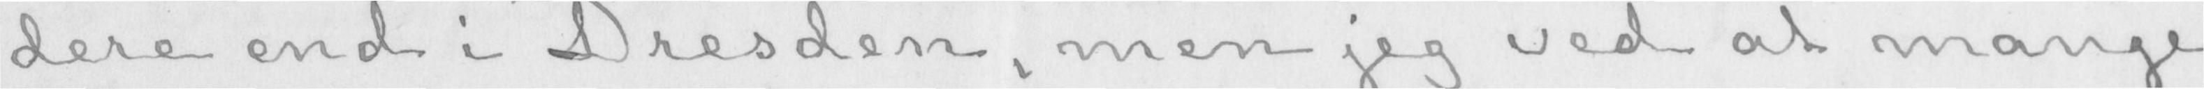dere end i Dresden, men jeg ved at mange
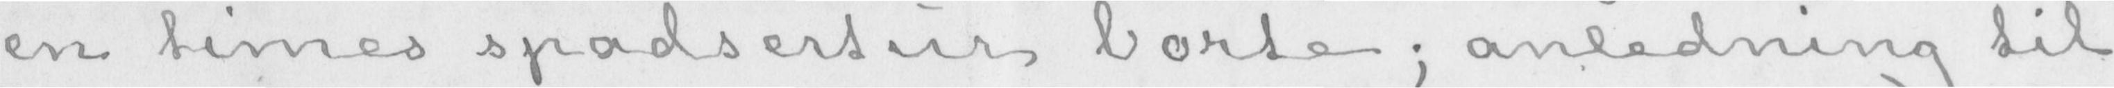en times spadsertur borte; anledning til
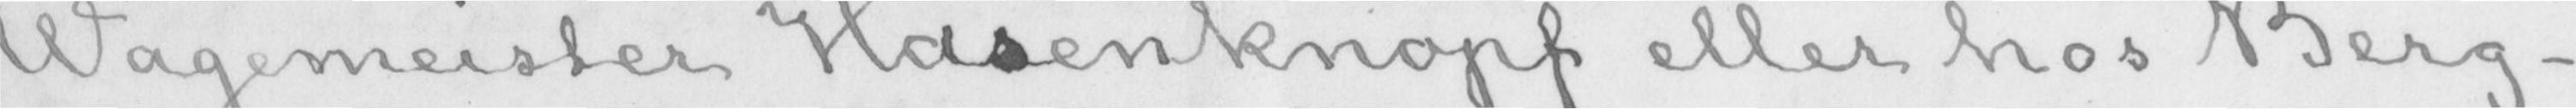Wagemeister Hasenknopf eller hos Berg-
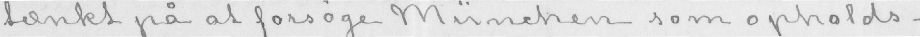tænkt på at forsøge München som opholds-
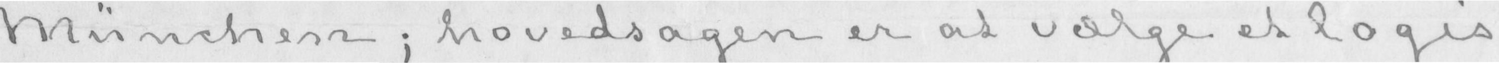München; hovedsagen er at vælge et logis
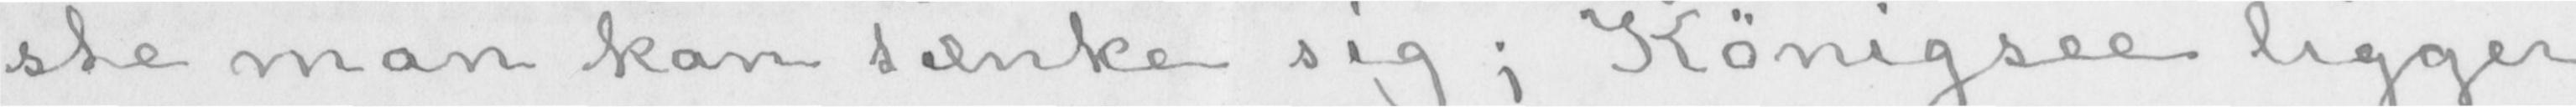ste man kan tænke sig; Königsee ligger
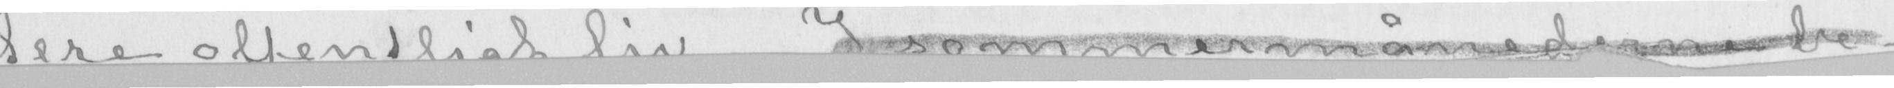tere offentligt liv. I sommermånederne be-
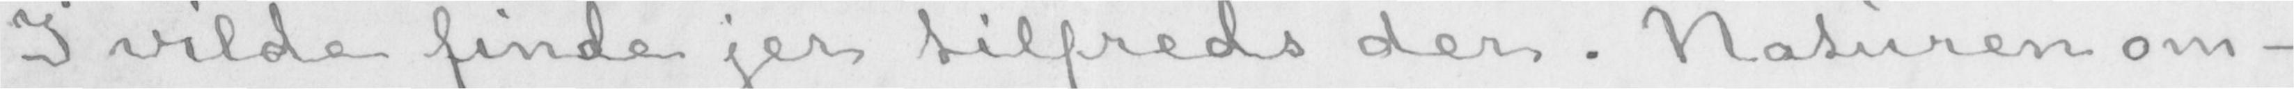I vilde finde jer tilfreds der. Naturen om-
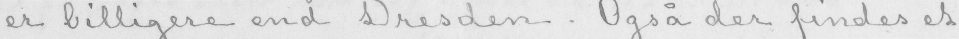er billigere end Dresden. Også der findes et
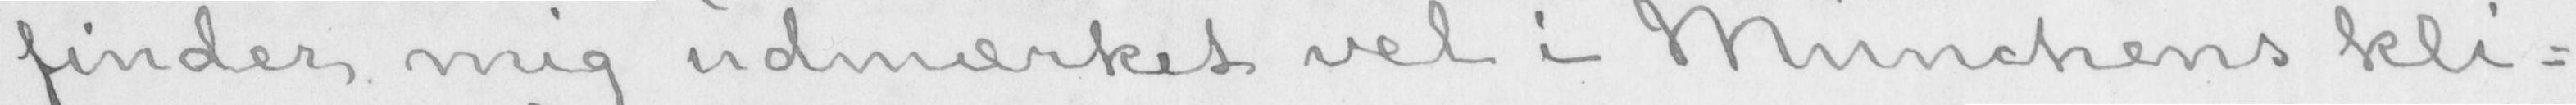finder mig udmærket vel i Münchens kli-
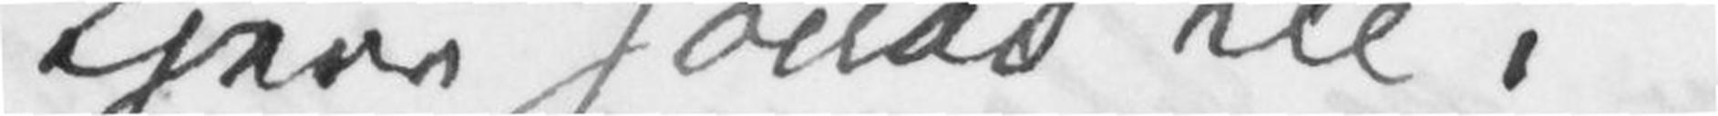Kjere Jonas Lie!
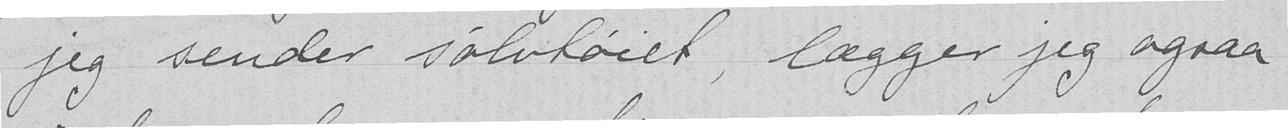jeg sender sølvtøiet, lægger jeg ogsaa
